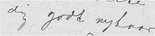dig godt nytaar!
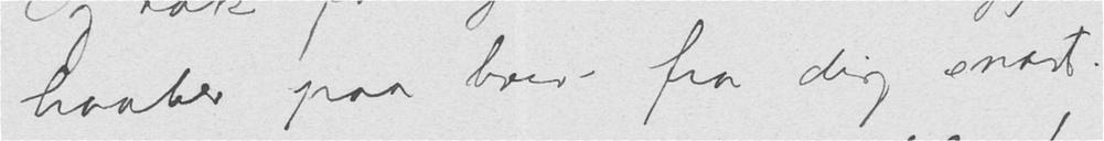haaber paa brev fra dig snart.
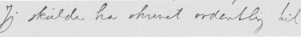Jeg skulde ha skrevet ordentlig til
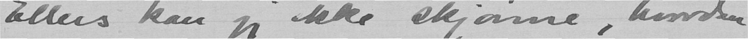Ellers kan jeg ikke skjønne, hvordan
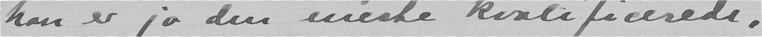han er jo den eneste kvalificerede,
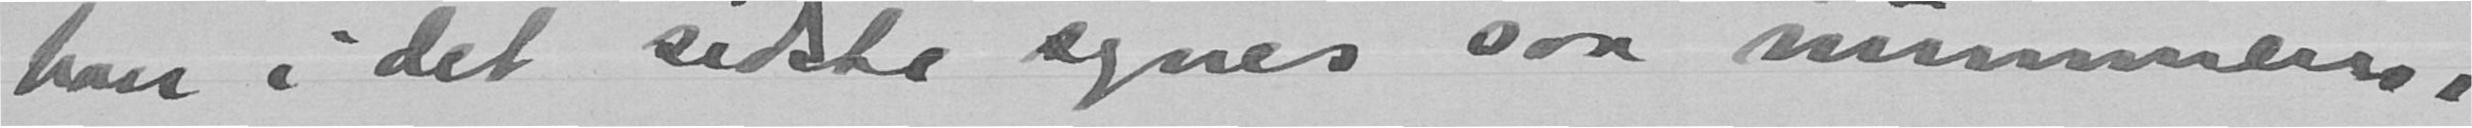han i det sidste synes som nummen,
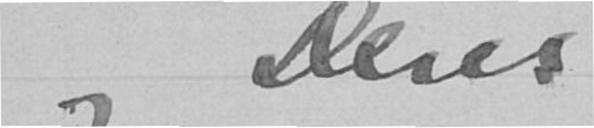og Deres
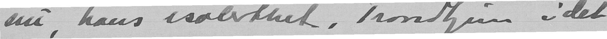nu, hans isolerthet, Trondhjem i det
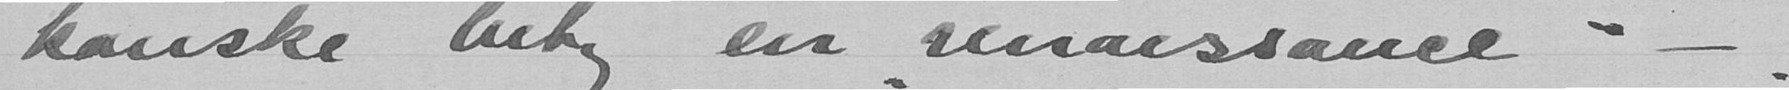kanske bety en "renaissance" -
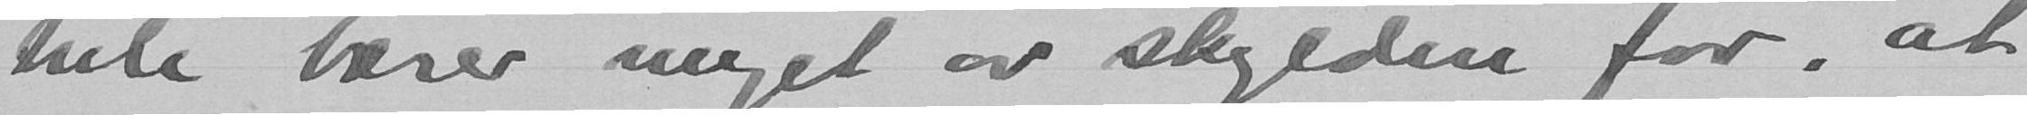hele bærer meget av skylden for, at
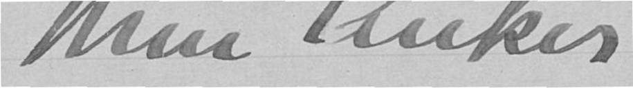Nini Anker
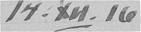14. XII. 16
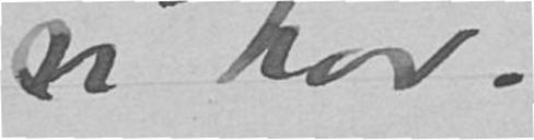vi har.
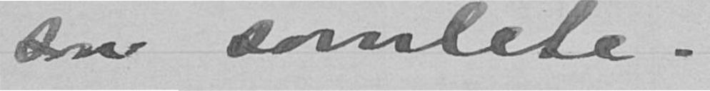som somlete.
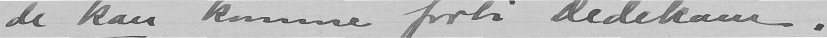de kan komme forbi Dedekam,
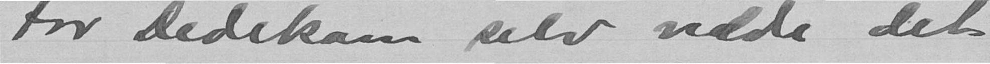For Dedekam selv vilde det
